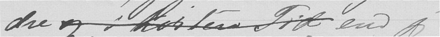dre og i kortere Tid end jeg
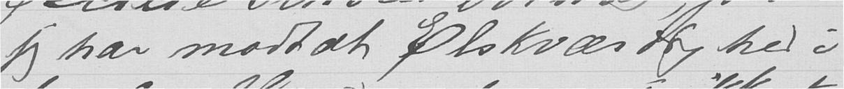jeg har modtat Elskværdighed i
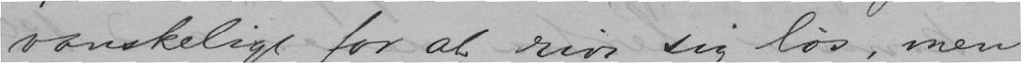vanskeligt for at rive sig løs, men
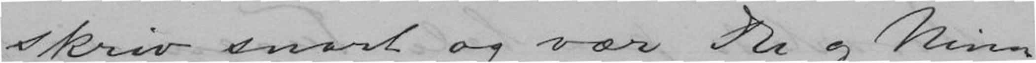skriv snart og vær Du og Nina
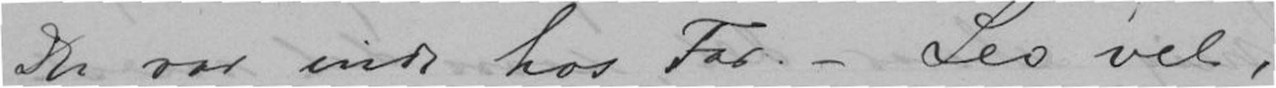Du var inde hos Far. - Lev vel,
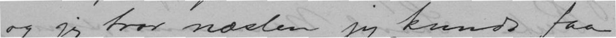og jeg tror næsten jeg kunde faa
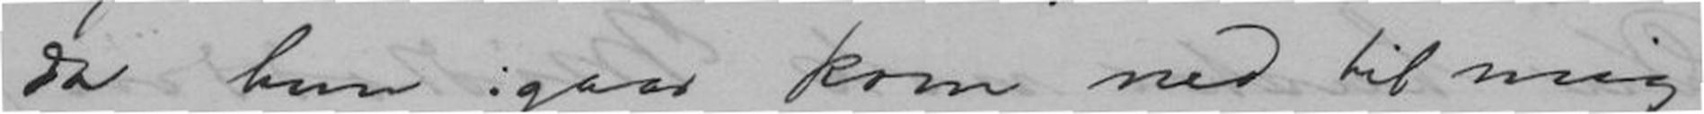da hun igaar kom ned til mig
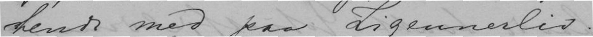hende med paa Zigeunerliv.
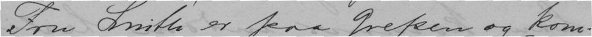Fru Smith er paa Grefsen og kom-
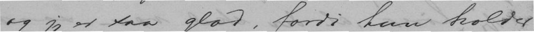og jeg er saa glad, fordi hun holder
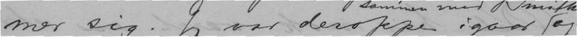mer sig. Jeg var deroppe igaar og
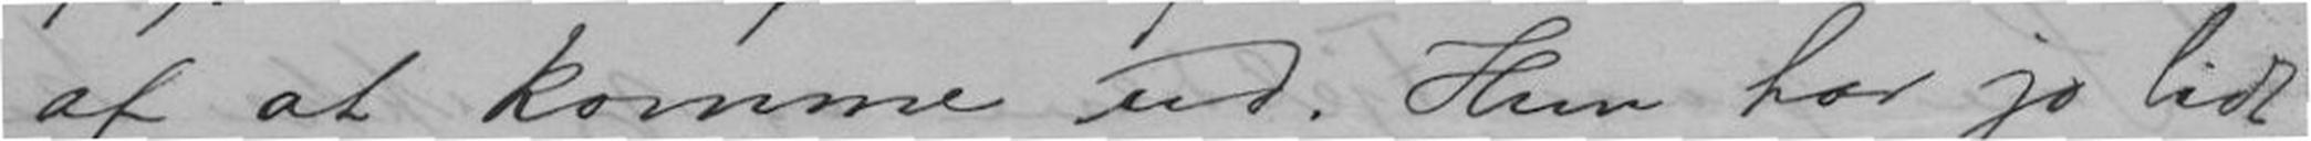af at komme ud. Hun har jo lidt
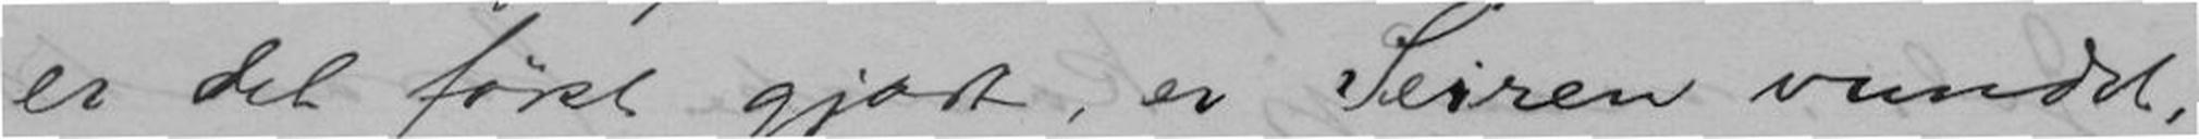er det først gjort, er Seiren vundet,
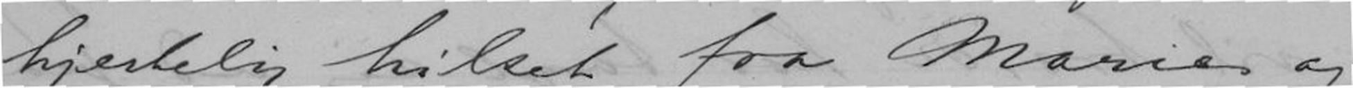hjertelig hilset fra Marie og
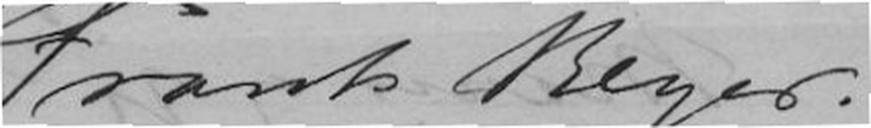Frants Beyer.
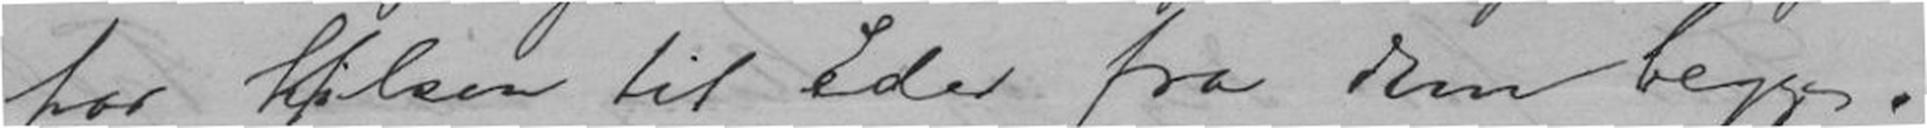har Hilsen til Eder fra dem begge.
